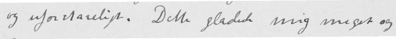og uforstaaeligt. Dette glædede mig meget og
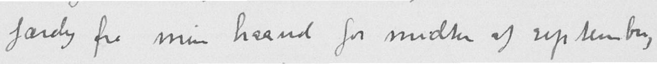færdig fra min haand før midten af september,
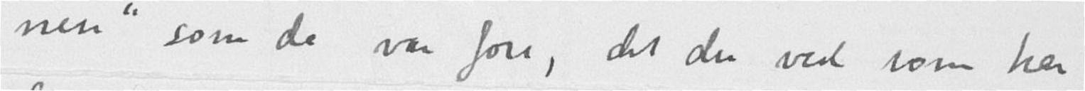nen" som da var fore, det du ved som har
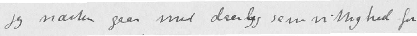jeg næsten gaar med daarlig samvittighed for
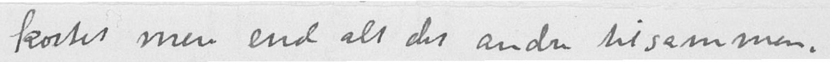kostet mere end alt det andre tilsammen.
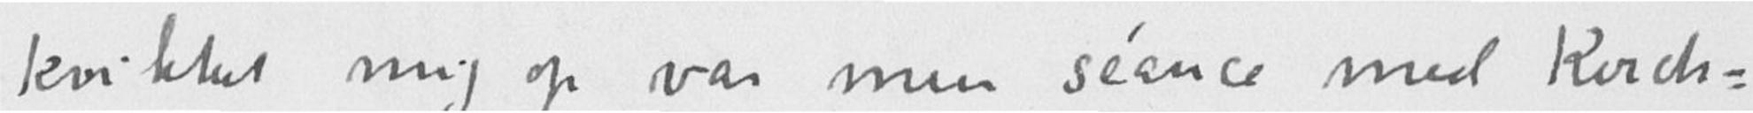kvikket mig op var min séance med Kirch-
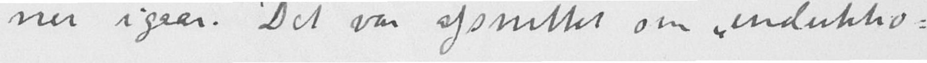ner igaar. Det var afsnittet om "induktio-
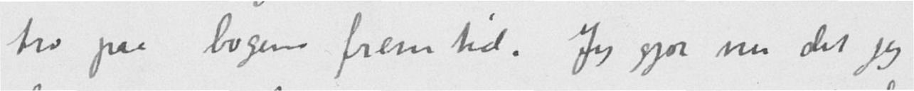tro paa bogens fremtid. Jeg gjør nu det jeg
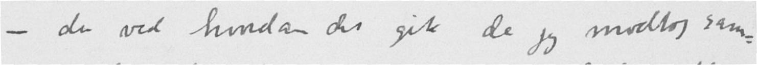- du ved hvordan det gik da jeg modtog sam-
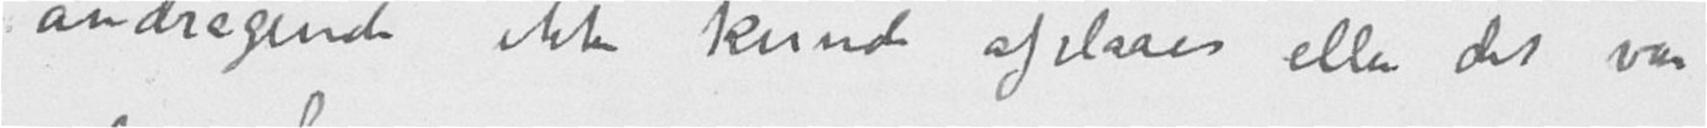andragende ikke kunde afslaaes eller det var
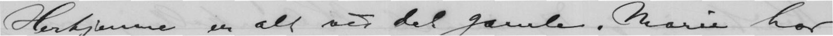Herhjemme er alt ved det gamle. Marie har
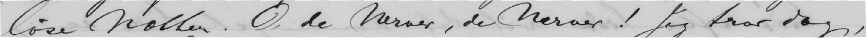løse Nætter. O de Nerver, de Nerver! Jeg tror dog,
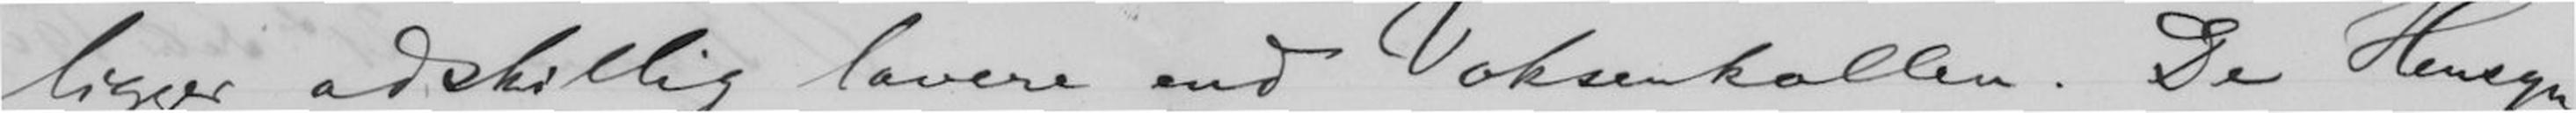ligger adskillig lavere end Voksenkollen. De Hensyn,
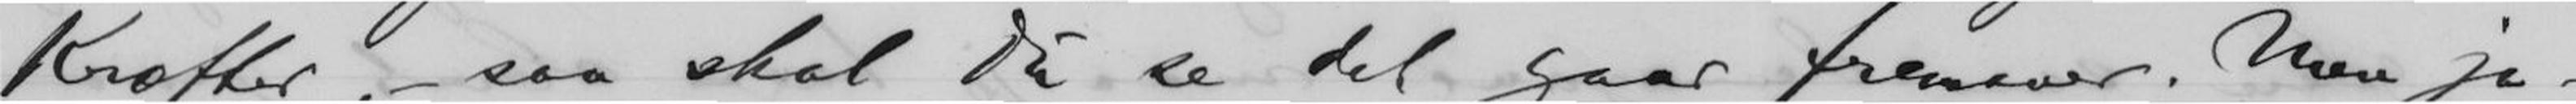Kræfter, - saa skal Du se det gaar fremover. Men ja-
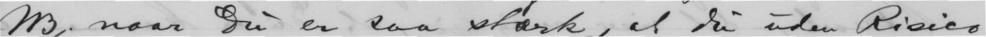NB; naar Du er saa stærk, at Du uden Risico
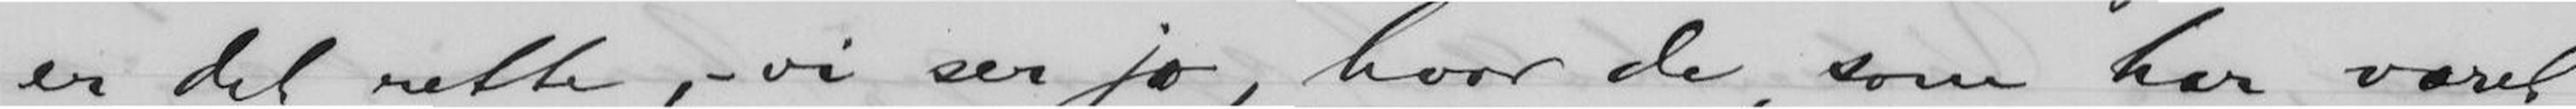er det rette, - vi ser jo, hvor de, som har været
